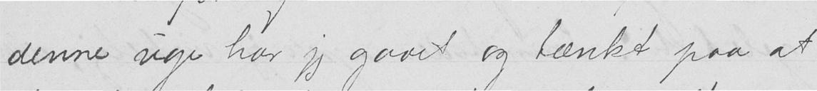denne uge har jeg gaaet og tænkt paa at
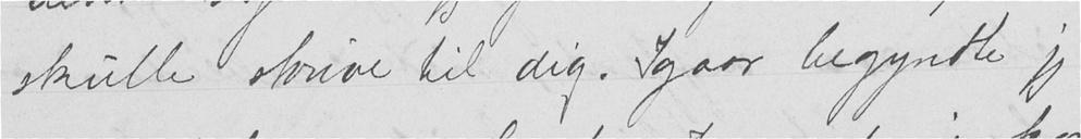skulle skrive til dig. Igaar begyndte jeg
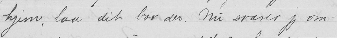hjem, laa dit brev der. Nu svarer jeg om-
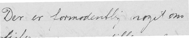Der er formodentlig noget om
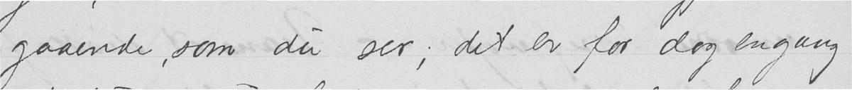gaaende, som du ser; det er for dog engang
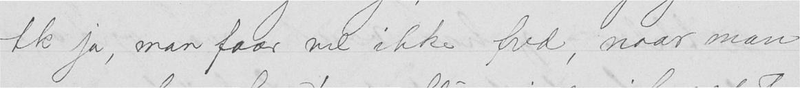Ak ja, man faar vel ikke fred, naar man
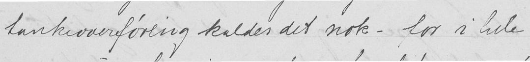tankeoverføring kaldes det nok - for i hele
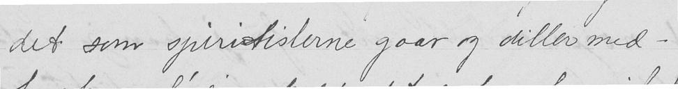det som spiritisterne gaar og diller med -
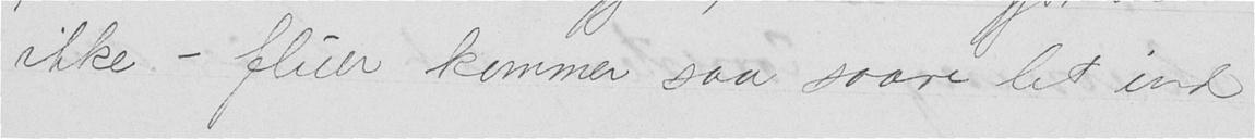ikke - fluer kommer saa svare let ind
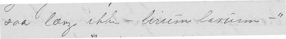saa længe ikke - lirum larum -"
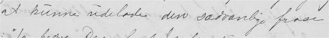at kunne udelade den sædvanlige frase
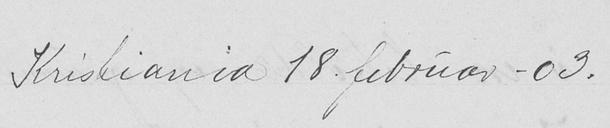Kristiania 18. februar -03.
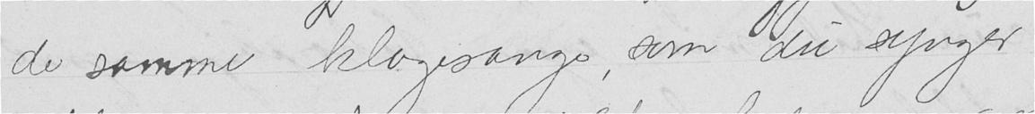de samme klagesange, som du synger
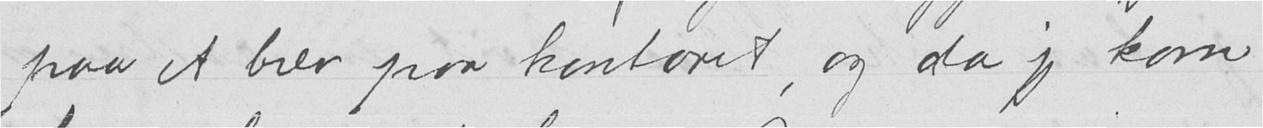paa et brev paa kontoret, og da jeg kom
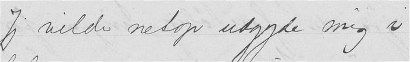Jeg vilde netop udgyde mig i
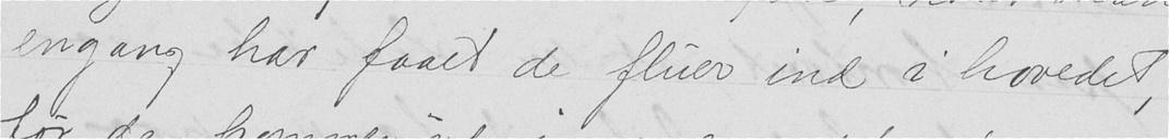engang har faaet de fluer ind i hovedet,
